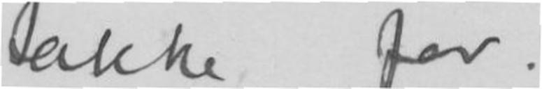takke for.
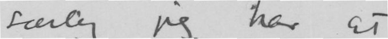særlig jeg har at
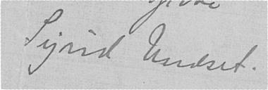Sigrid Undset.
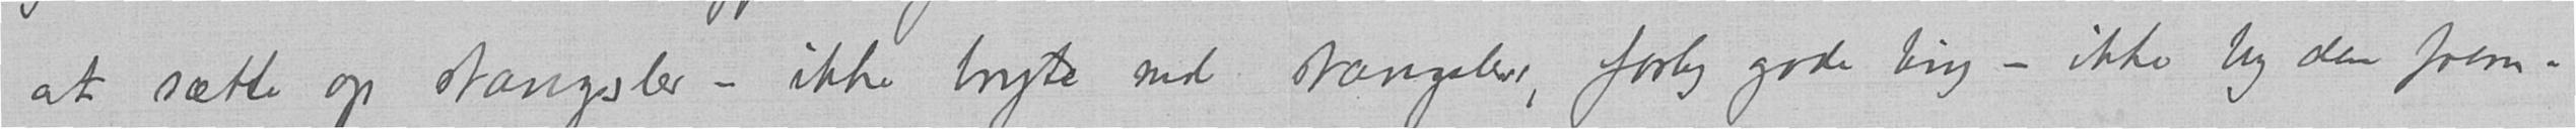at sætte op stængsler – ikke bryte ned stængsler, forby gode ting – ikke by dem frem.
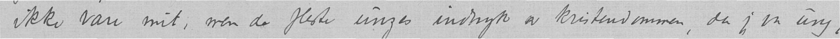ikke bare mit, men de fleste unges indtryk av kristendommen, da jeg var ung,
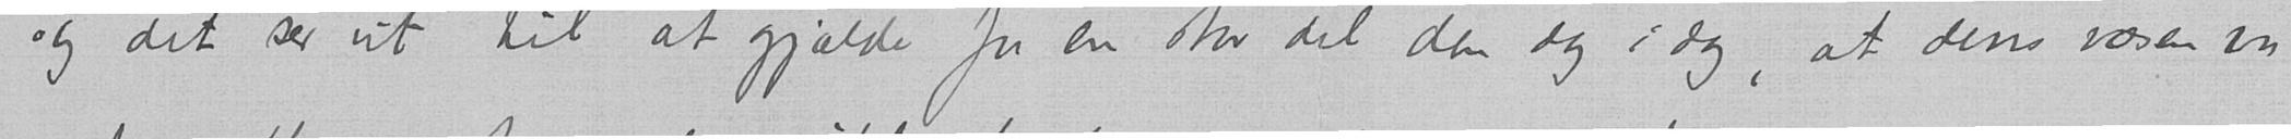og det ser ut til at gjælde for en stor del den dag i dag, at dens væsen var
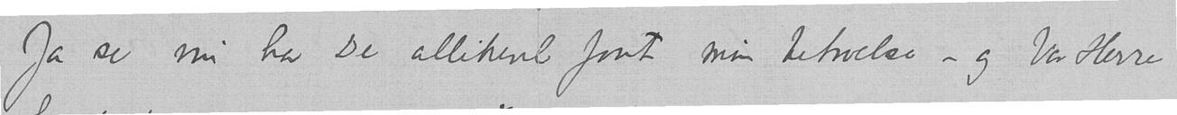Ja se nu har De allikevel faat min betroelse – og Vor Herre
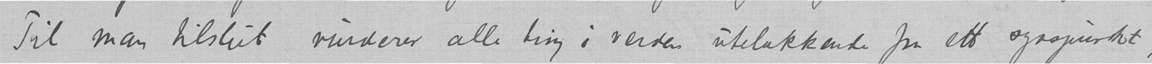Til man tilslut vurderer alle ting i verden utelukkende fra ett synspunkt,
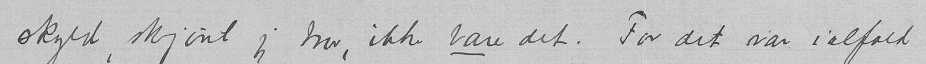skyld, skjønt jeg tror, ikke bare det. For det var ialfald
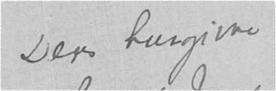Deres hengivne
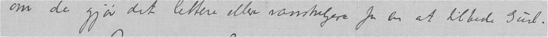om de gjør det lettere eller vanskeligere for en at tilbede Gud.
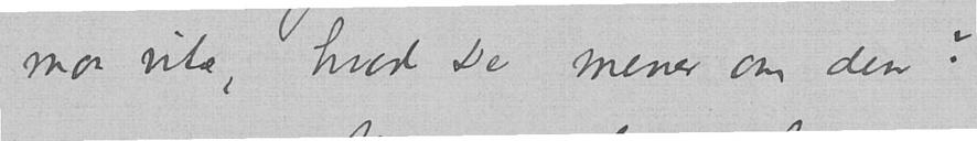maa vite, hvad De mener om den?
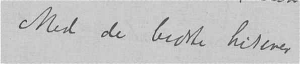Med de bedste hilsener
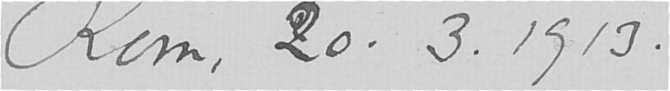Rom, 20.3.1913.
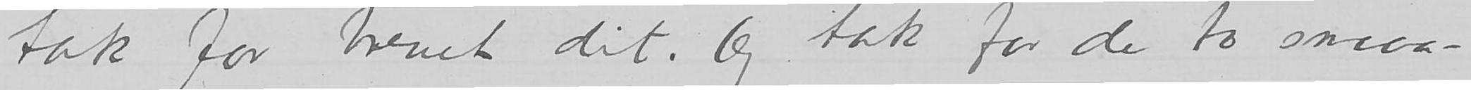tak for brevet dit. Og tak for de to smaa-
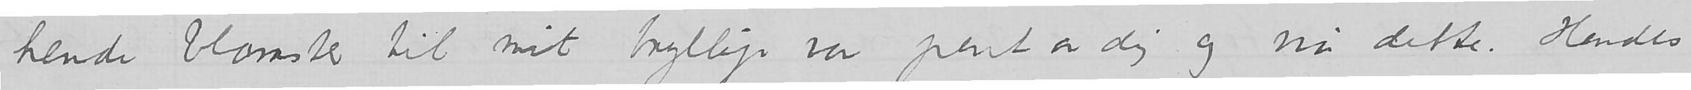hende blomster til mit bryllup var pent av dig og nu dette. Hendes
